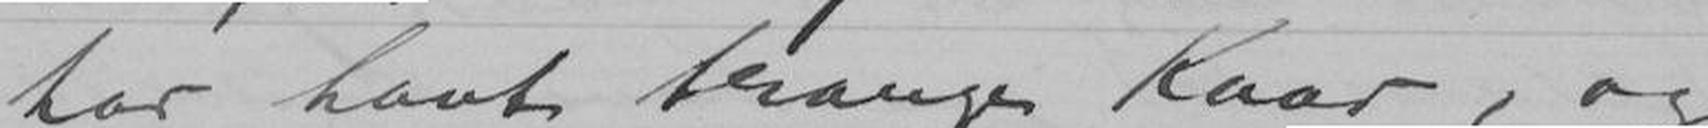har havt trange Kaar, og
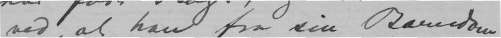ved, at han fra sin Barndom
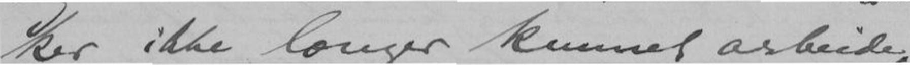ker ikke længer kunnet arbeide,
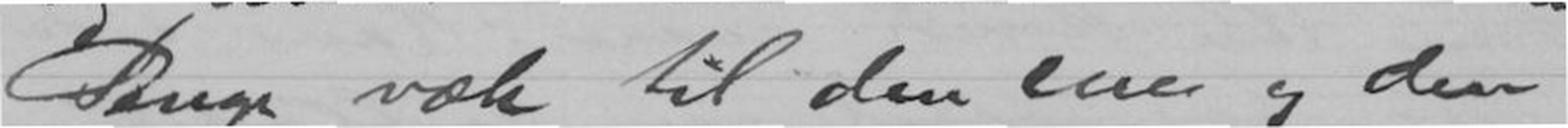Penge væk til den ene og den
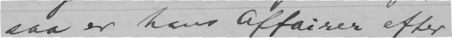saa er hans Affairer efter-
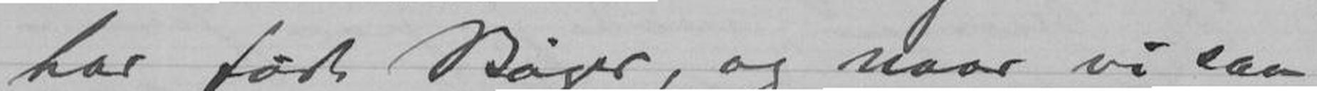har ført Bøger, og naar vi saa
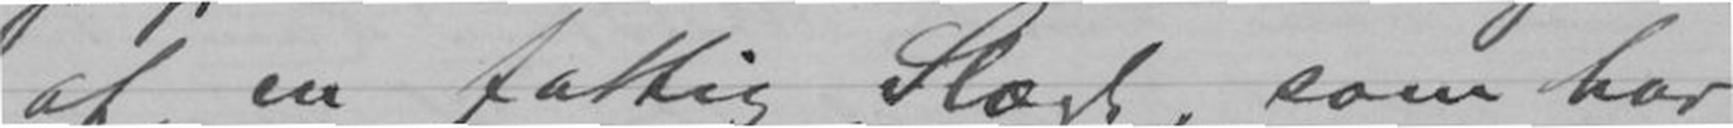af en fattig Slægt, som har
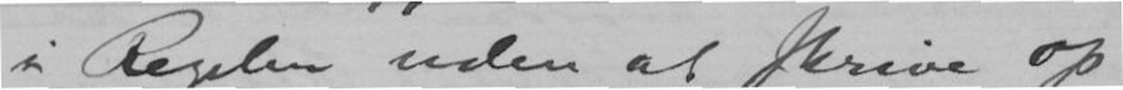i Regelen uden at skrive op,
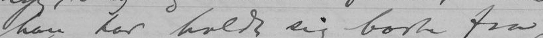han har holdt sig borte fra
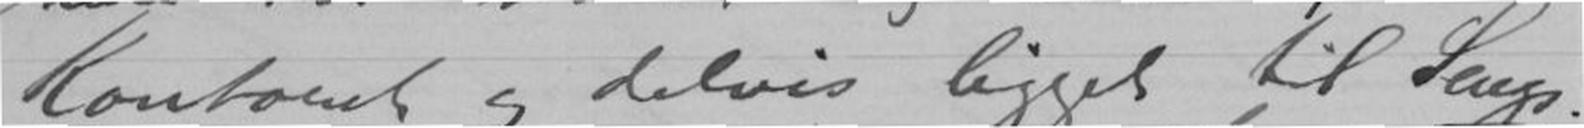Kontoret og delvis ligget til Sengs.
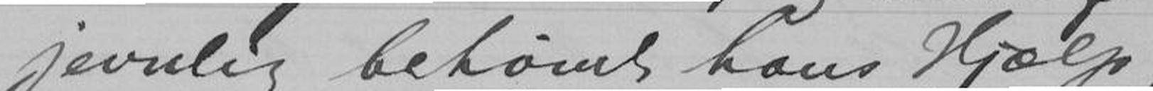jevnlig behøvet hans Hjælp,
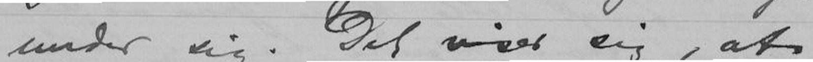under sig. Det viser sig, at
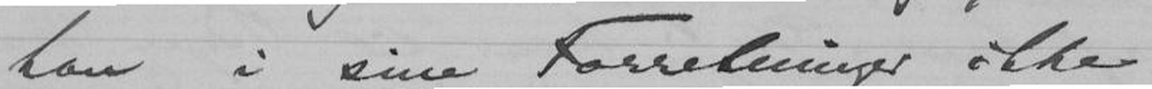han i sine Forretninger ikke
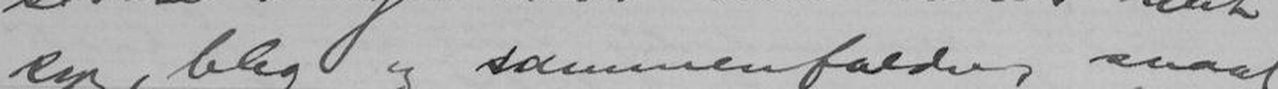syg, bleg og sammenfalden, saaat
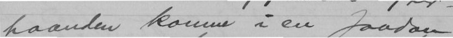haanden komme i en saadan
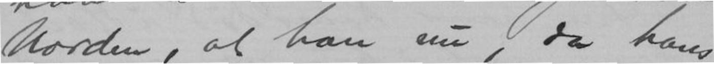Uorden, at han nu, da hans
